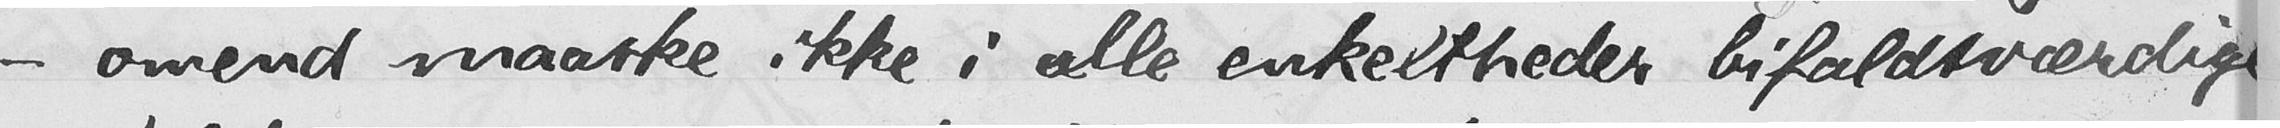- omend maaske ikke i alle enkeltheder bifaldsværdig
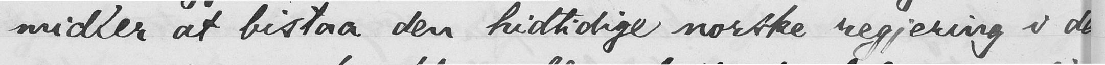midler at bistaa den hidtidige norske regjering i d
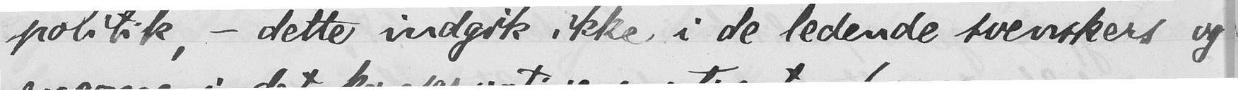politik, - dette indgik ikke i de ledende svenskers og
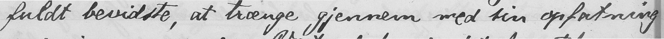fuldt bevidste, at trænge gjennem med sin opfatning
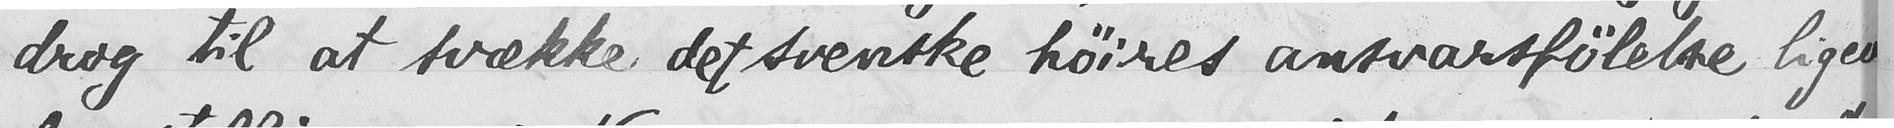drog til at svække det svenske høires ansvarsfølelse lige
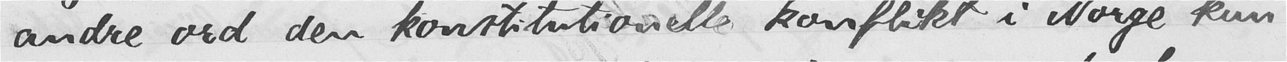andre ord den konstitutionelle konflikt i Norge kun
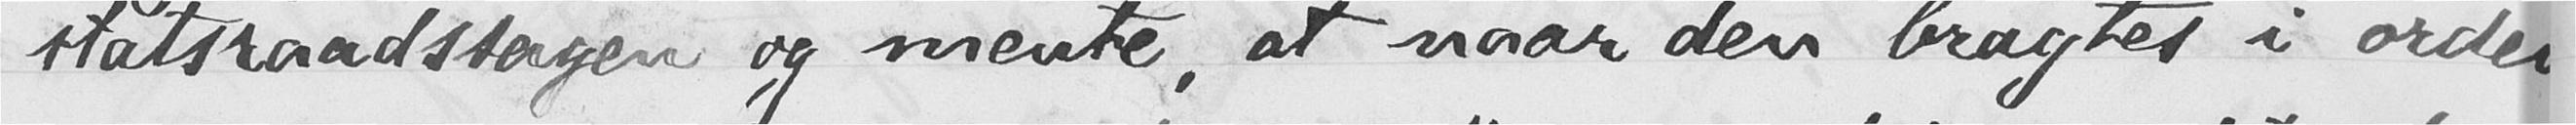statsraadssagen og mente, at naar den bragtes i orde
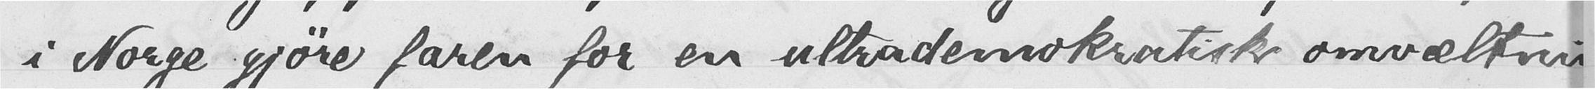i Norge gjøre faren for en ultrademokratisk omvæltning
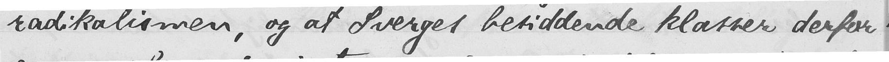radikalismen, og at Sverges besiddende klasser derfor
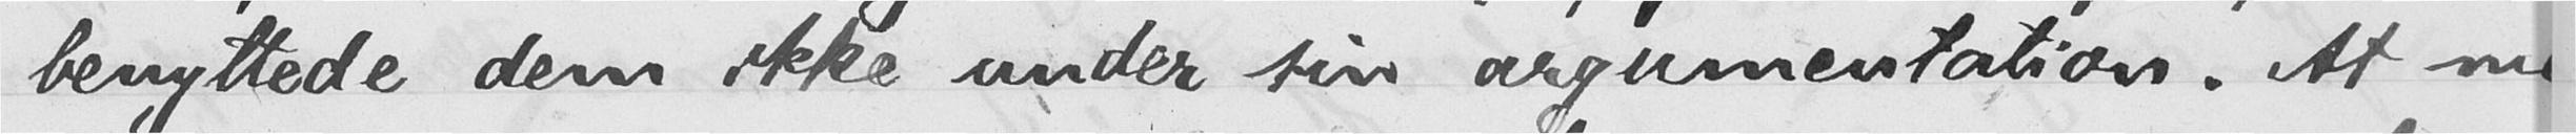benyttede dem ikke under sin argumentation. At m
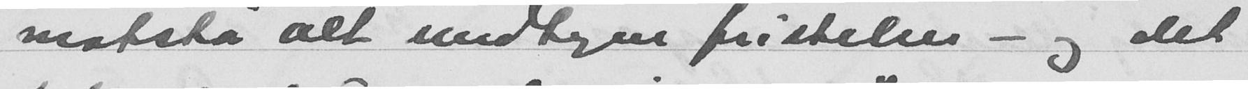motstå alt undtagen fristelser - og det
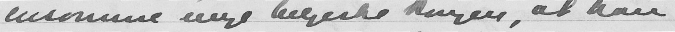ensomme unge belgiske kongen, at han
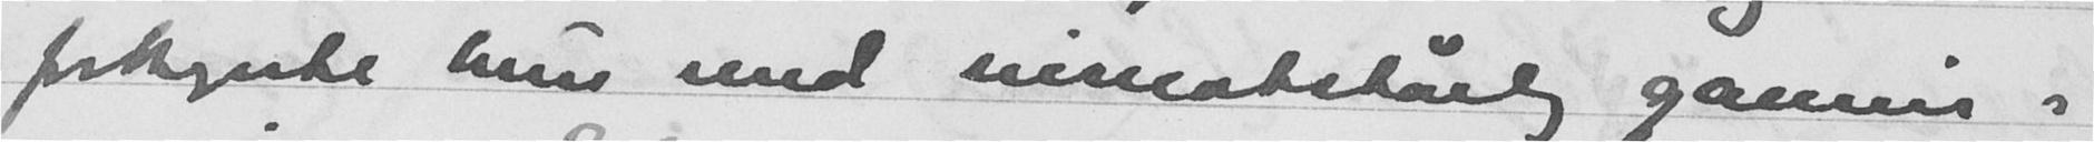forkynte hun med uimotståelig gamm-
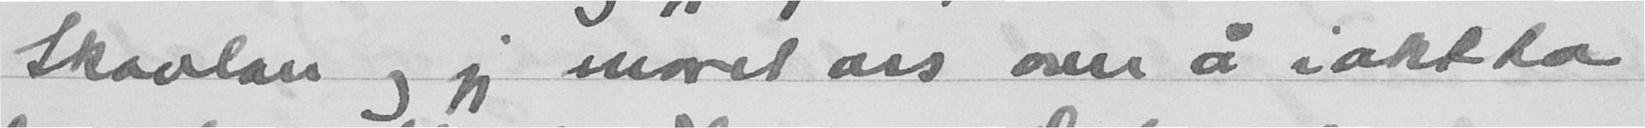Skavlan og jeg moret oss om å iakta
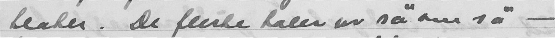teater. De fleste taler var så som så -
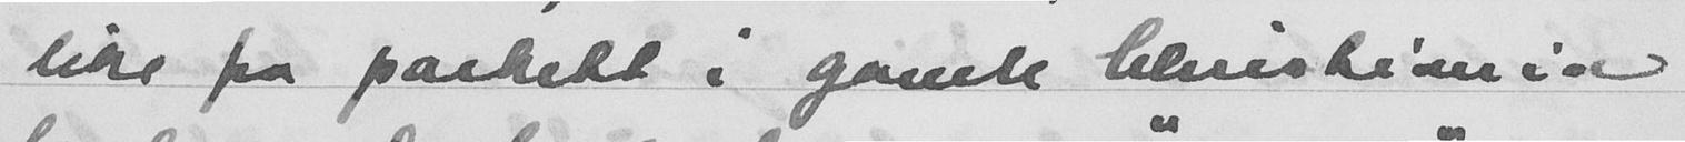like fra parkett i gamle Christiania
